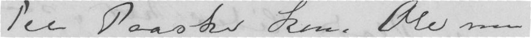Til Paaske kom Ole me
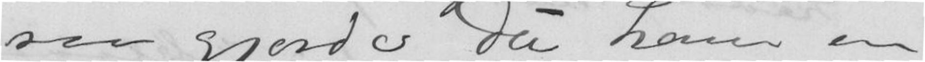saa gjorde Du ham en
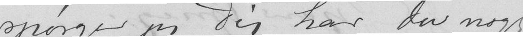spørger jeg Dig har Du noget
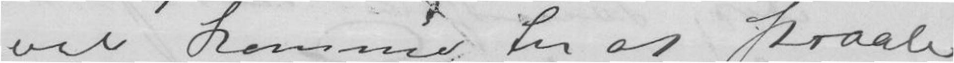vil komme til at straale
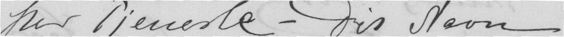stor Tjeneste - Dit Navn
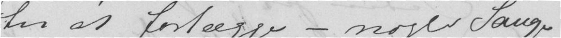til at forlægge - nogle Sange
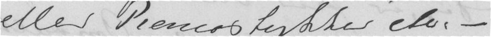eller Pianostykker etc. -
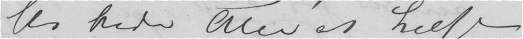Vi bede Alle at hilse
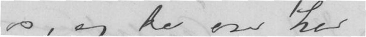os, og De ere her
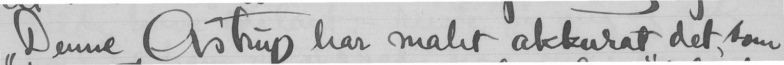"Denne Astrup har malet akkurat det, som
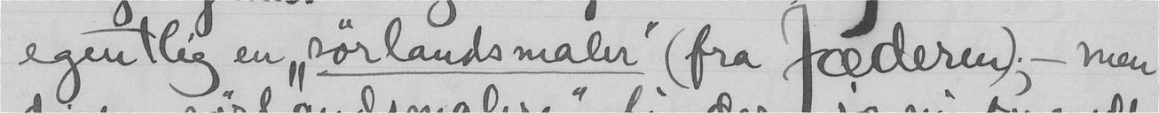egentlig en "sørlandsmaler" (fra Jæderen); - men
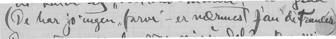(De har jo ingen "farve" - er nærmest Jau di Frances)
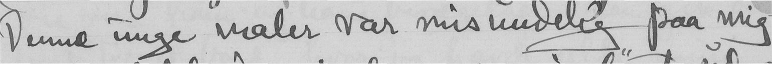Denne unge maler var misundelig paa mig
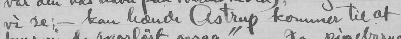vi se; - kan hænde Astrup kommer til at
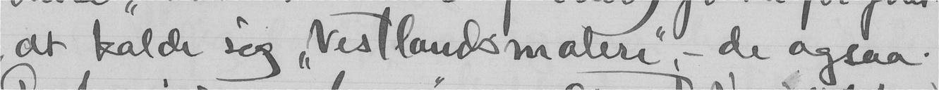at kalde sig "vestlandsmalere" - de ogsaa.
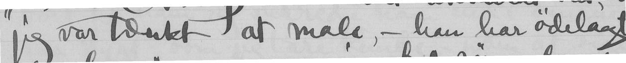jeg var tænkt at male, - han har ødelagt
